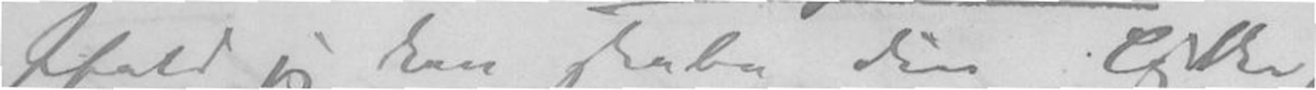Ifald jeg kan skabe din Lykke,
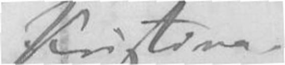Kristina.
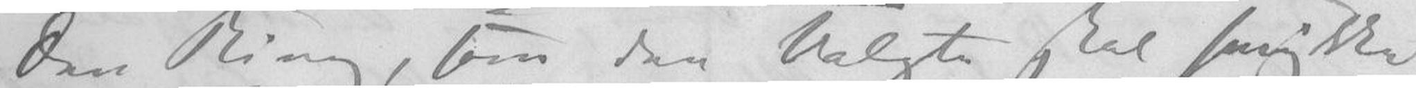Den Ring, som den Valgte skal Smykke
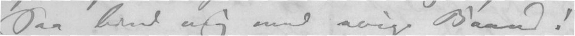Saa bind mig med evig Baand!
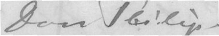Don Philip.
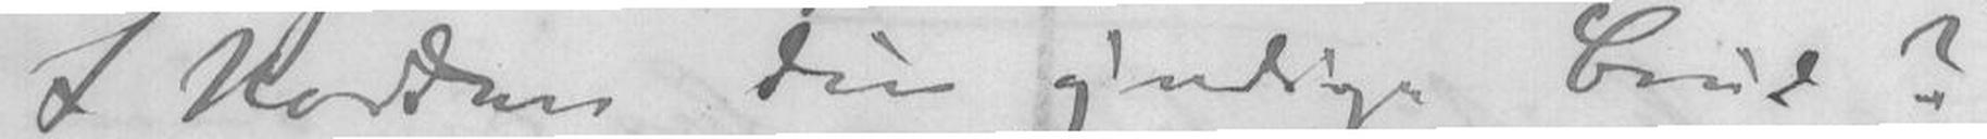I Norden din yndige Brud?
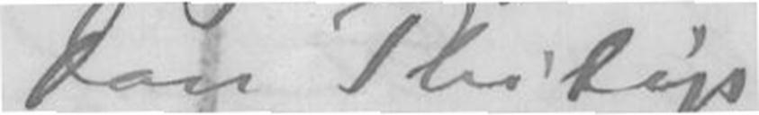Don Philip
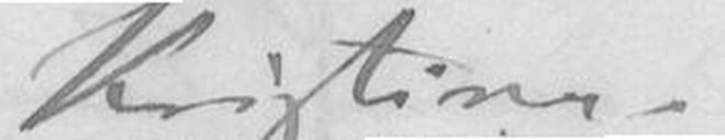Kristina.
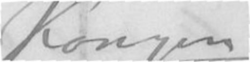Kongen
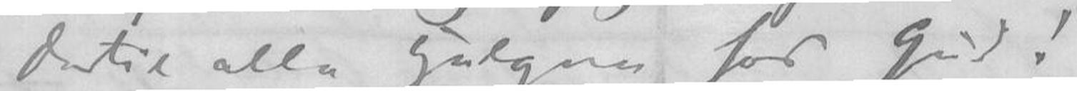Dertil alle Helgen hos Gud!
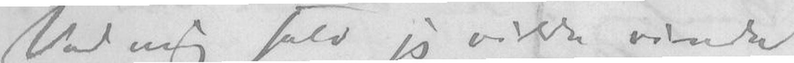Vad mig selv jeg vilde vinde
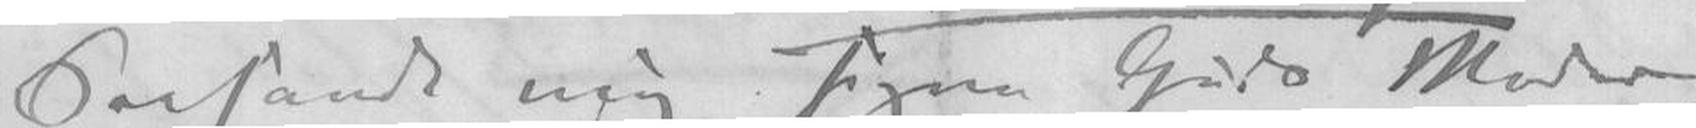Saasandt mig signe Guds Moder,
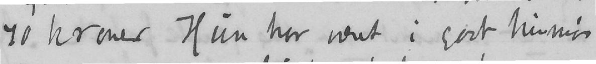70 kroner Hun har været i godt humør
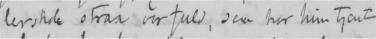lerskole strax var fuld, saa har hun tjent
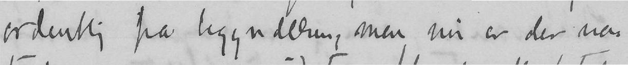ordentlig fra begyndelsen, men nu er der na-
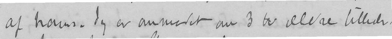af ham. Jeg er anmodet om 3 bi ældre billeder,
